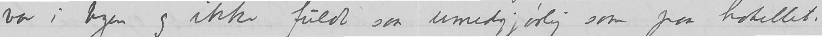var i byen og ikke fuldt saa umedgjørlig som paa hotellet.
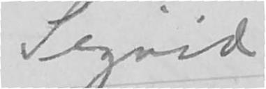Sigrid.
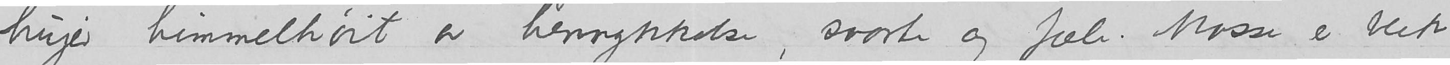hujer himmelhøit av henrykkelse, svarte og fæle. Mosse er blek
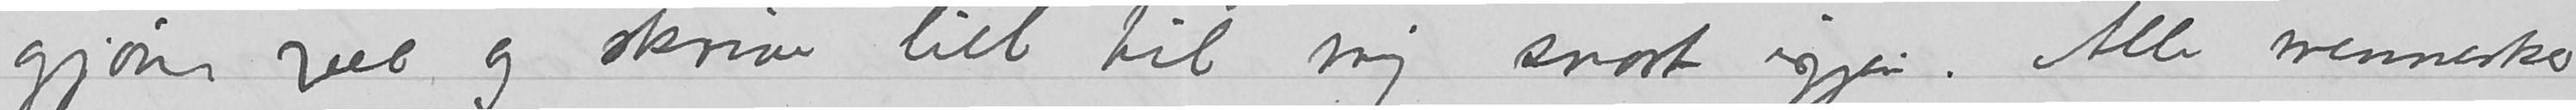gjøre vel og skrive litt til mig snart igjen. Alle mennesker
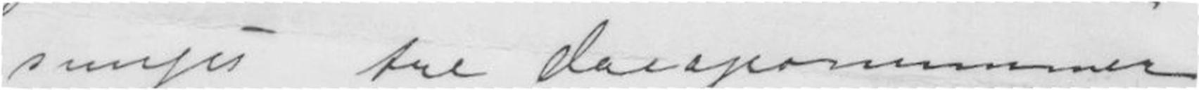sunget tre dacaponummer
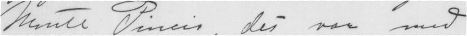Monte Pincio, det var med
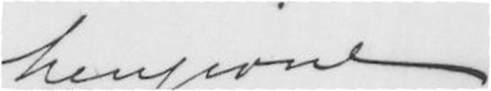hengivne
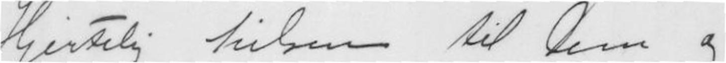Hjertelig hilsen til Dem og
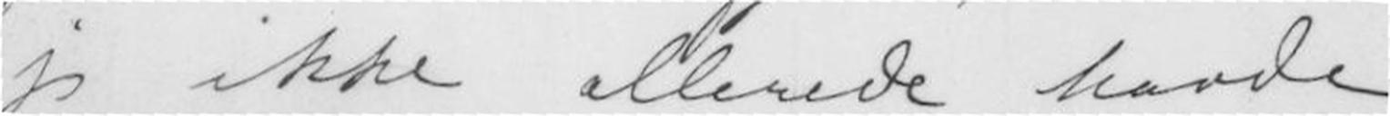jeg ikke allerede havde
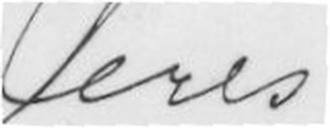Deres
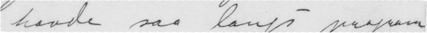jeg havde saa langt program
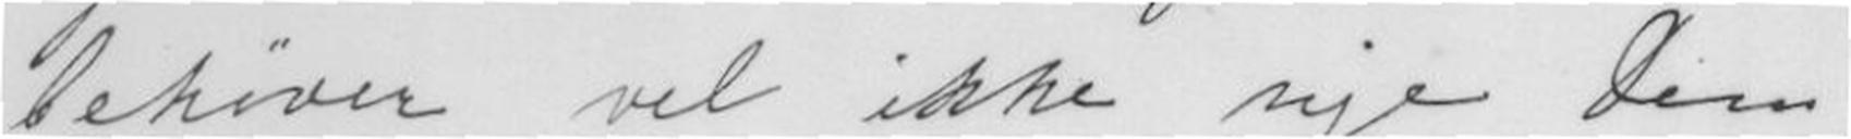behøver vel ikke sige Dem,
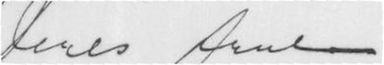Deres frue
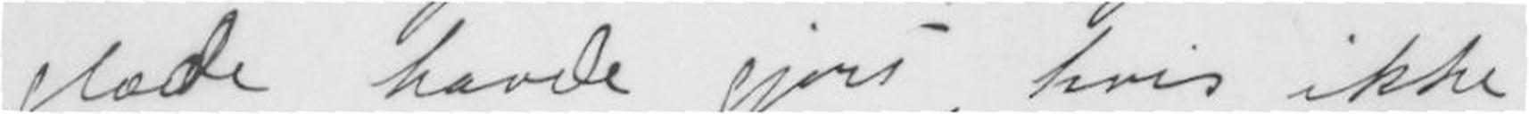glæde havde gjort, hvis ikke
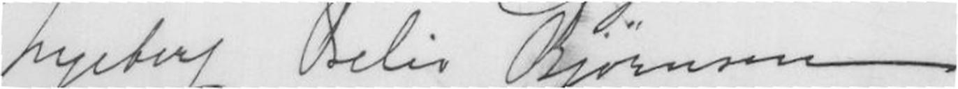Ingeborg Oselio Bjørnson
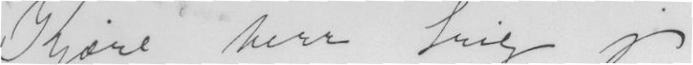Kjære herr Grieg jeg
